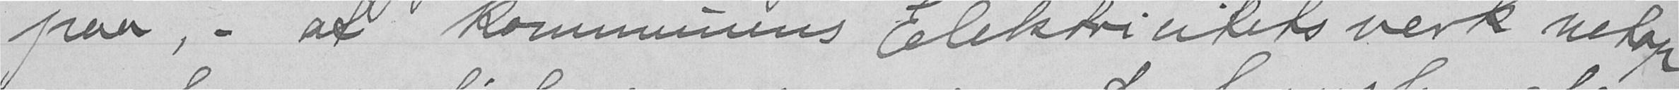paa, - at kommunens Elektricitetsverk netop
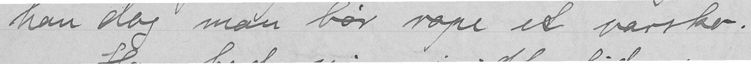han dog man bør rope et varsko.
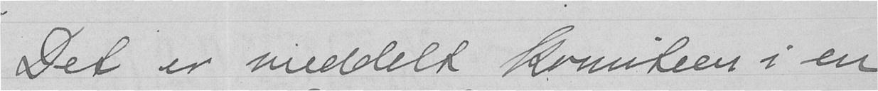Det er meddelt komiteen i en
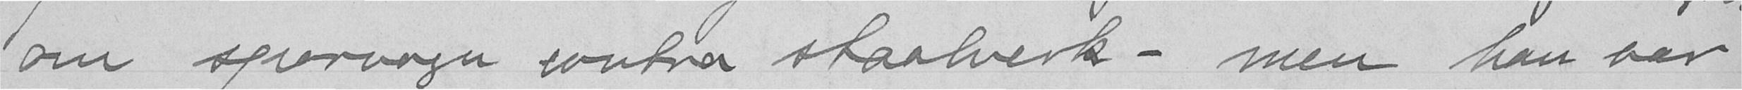om sporvogn contra staalverk - men han var
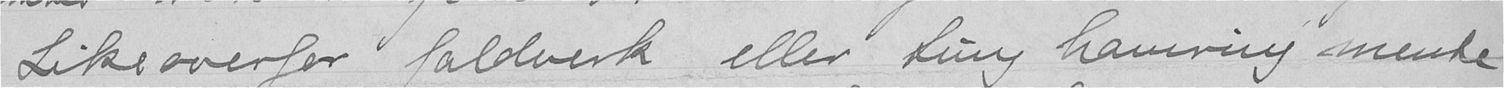Likeoverfor faldverk eller tung hamring mente
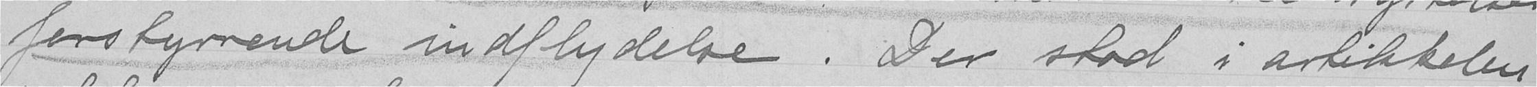forstyrrende indflydelse. Der stod i artikkelen
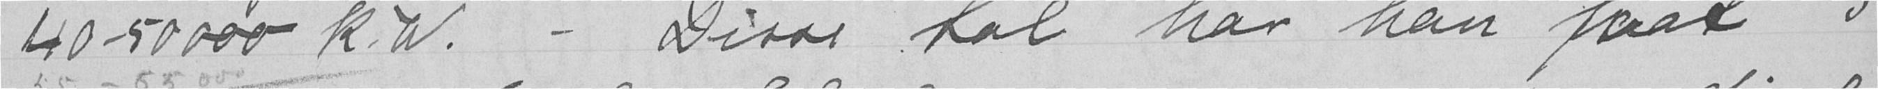40-50000 K.W. - Disse tal har han faat
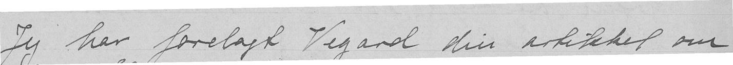Jeg har forelagt Vegard din artikkel om
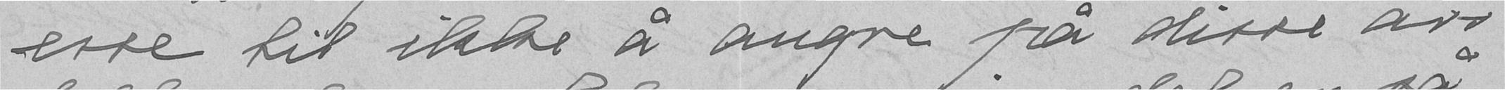esse til ikke å angre på disse års
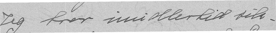Jeg tror imidlertid sik
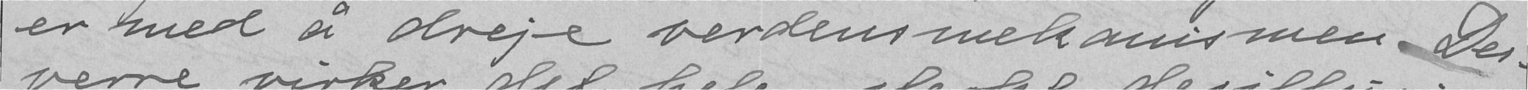er med å dreje verdensmekanismen. Des-
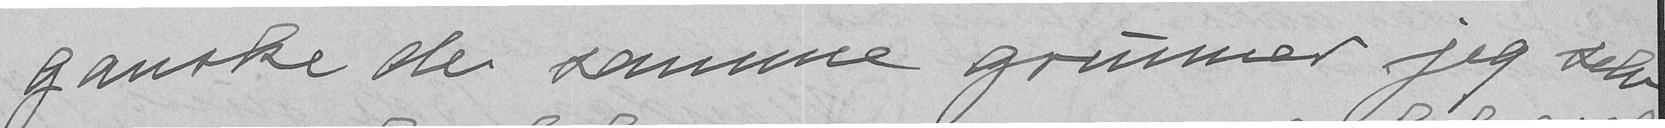ganske de samme grunner jeg selv
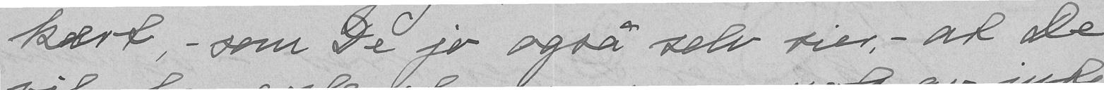kert, - som De jo også selv sier,- at De
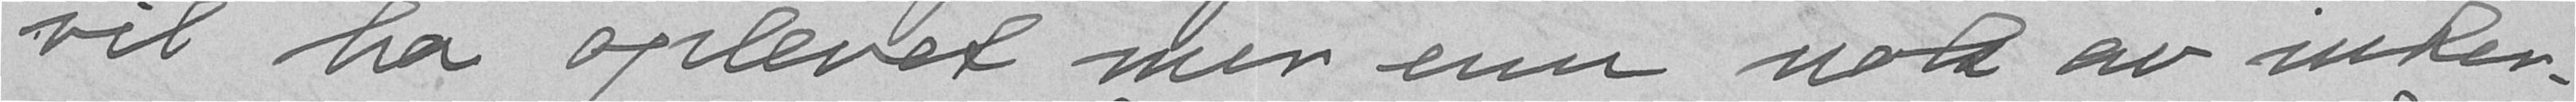vil ha oplevet mer enn nok av inter-
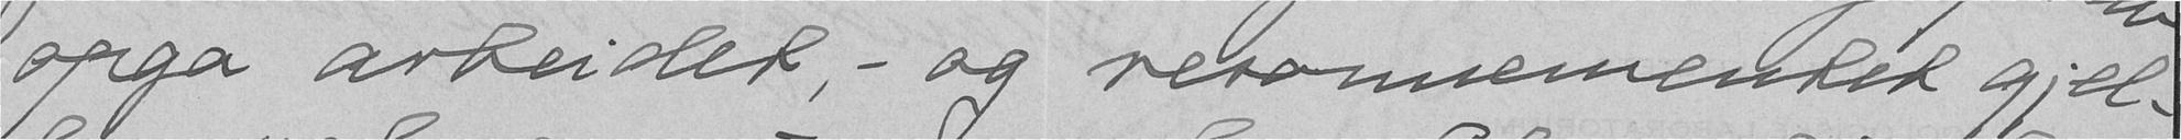opga arbeidet, - og resonnementet gjel-
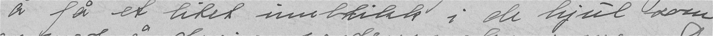å få et litet innblikk i de hjul som
